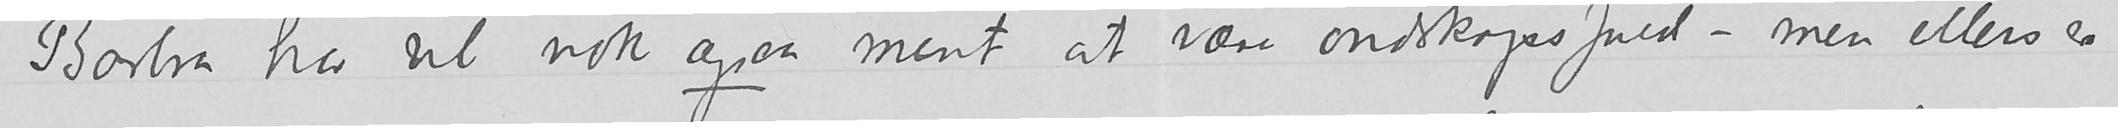Barbra har vel nok ogsaa ment at være ondskapsfuld – men ellers er
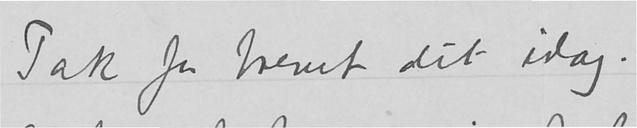Tak for brevet dit idag.
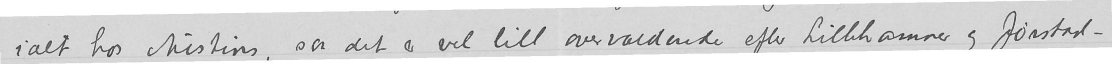i alt hos Austins, saa det er vel litt overvældende efter Lillehammer og Jørstad-
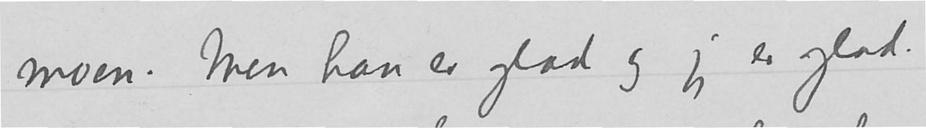moen. Men han er glad og jeg er glad.
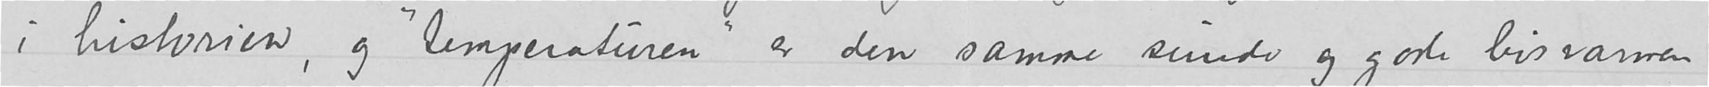i historien, og «temperaturen» er den samme sunde og gode livsvarmen
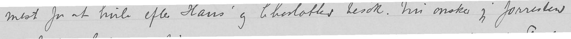mest for at hvile efter Hans og Charlottes besøk. Nu ønsker jeg forresten
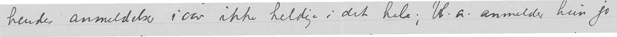hendes anmeldelser iaar ikke heldige i det hele; bl.a. anmelder hun jo
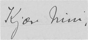Kjære Nini,
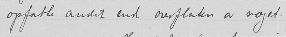opfatte andet end overflaten av noget.
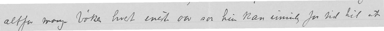altfor mange bøker hvert eneste aar saa hun kan umulig faa tid til at
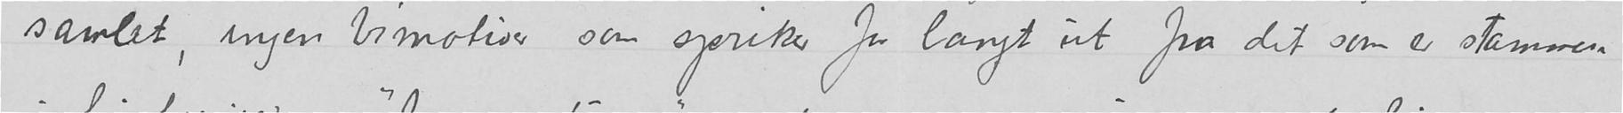samlet, ingen bimotiver som spriker for langt ut fra det som er stammen
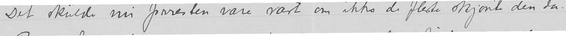Det skulde nu forreste være rart om ikke de fleste skjønte den da.
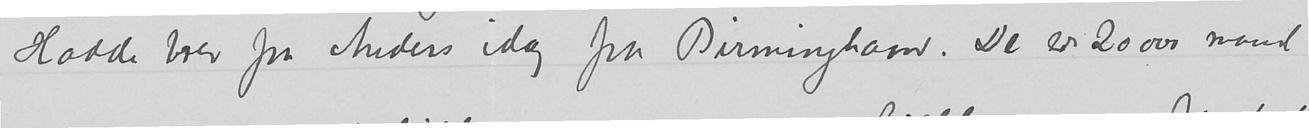Hadde brev fra Anders idag fra Birmingham. De er 20 000 mand
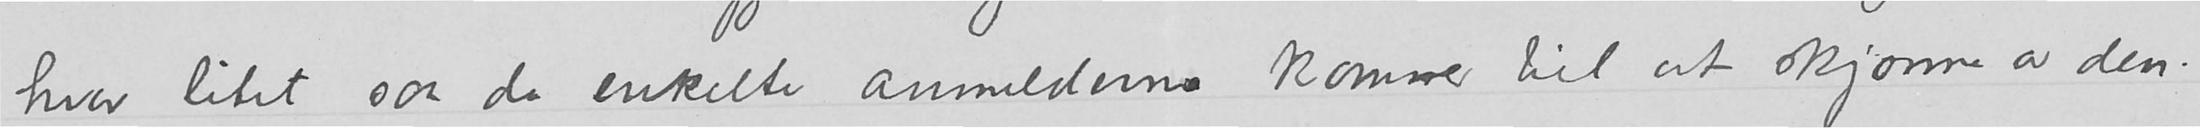hvor litet som de enkelte anmelderne kommer til at skjønne av den.
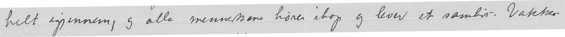helt igjennem og alle menneskene hører ihop og lever et samliv. Vakker
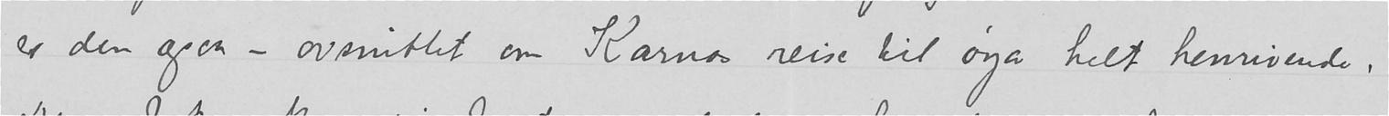er den ogsaa – avsittet om Karnas reise til øya helt henrivende.
